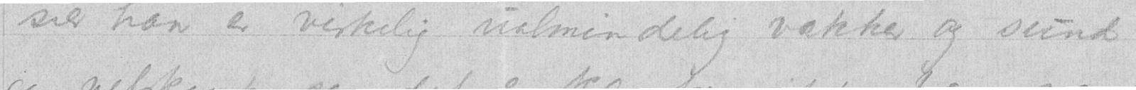sier han er virkelig ualmindelig vakker og sund
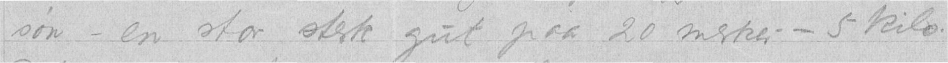søn - en stor sterk gut paa 20 merker - 5 kilo.
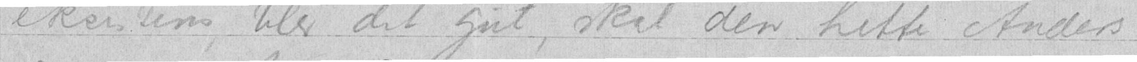eksistens blev det gut, skal den hette Anders
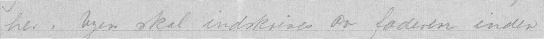her i byen skal indskrives av faderen inden
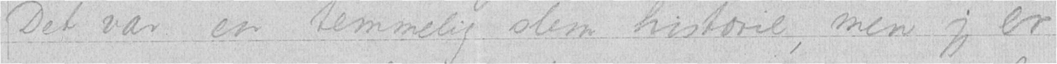Det var en temmelig slem historie men jeg er
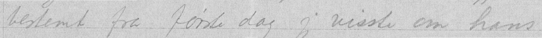bestemt fra første dag jeg visste om hans
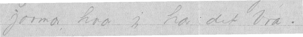jormor, hvor jeg har det bra.
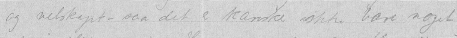og velskapt , saa det er kanske ikke bare noget
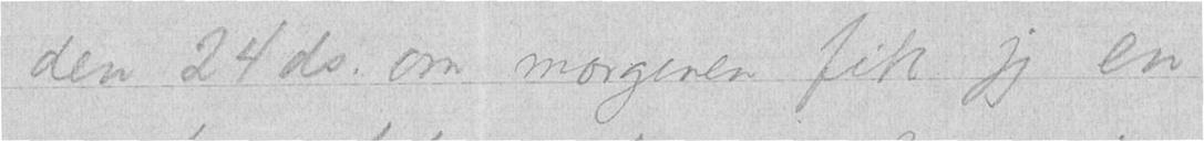den 24de. om morgenen fik jeg en
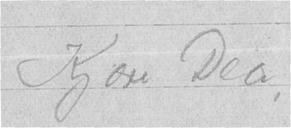Kjære Dea,
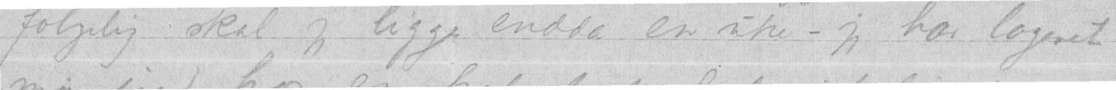følgelig, skal jeg ligge endda en uke - jeg har logeret
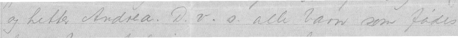og hetter Andrea. D.v.s. alle barn som fødes
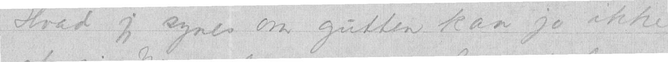Hvad jeg synes om gutten kan jo ikke
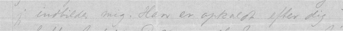jeg indbilder mig. Han er opkaldt efter dig
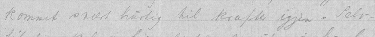kommet svært hurtig til kræfter igjen - Selv-
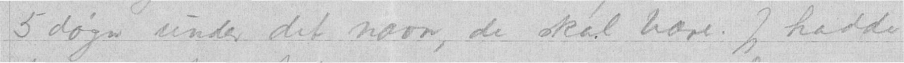5 døgn under det navn, de skal bære. Jeg hadde
